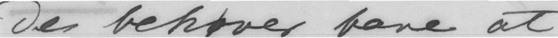De behøver bare at
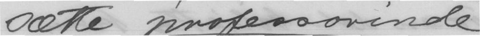sætte professorinde
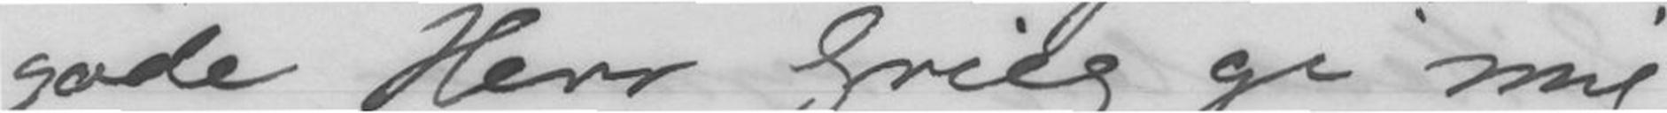gode Herr Grieg gi mig
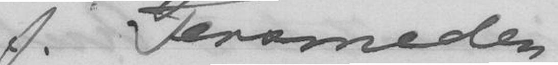f. Tersmeden
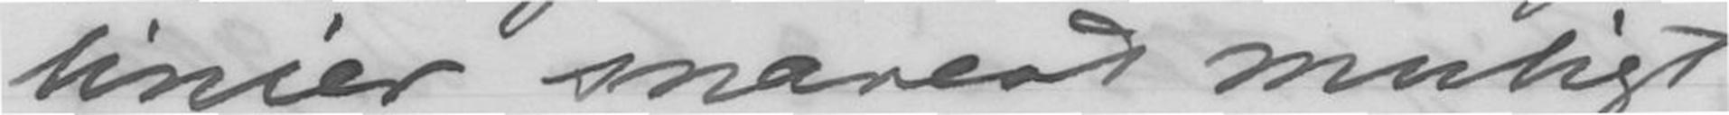linier snarest muligt.
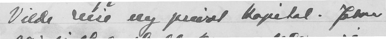Vilde reise ny privat kapital. Johan
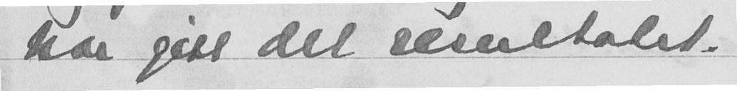har gitt det resultatet.
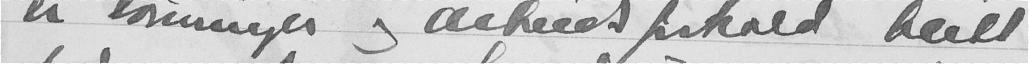er lønninger og arbeidsforhold blitt
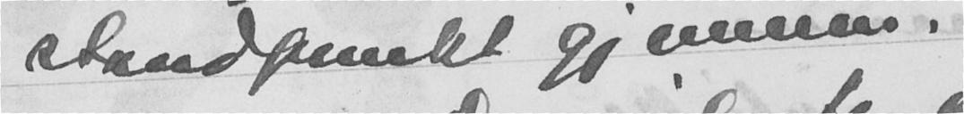standpunkt gjennom.
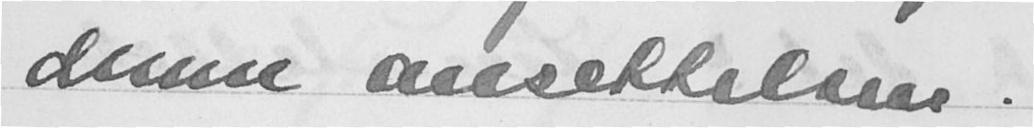denne ansettelsen.
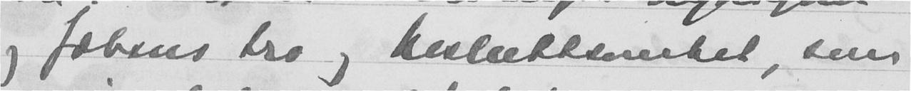og Johans tro og besluttsomhet, som
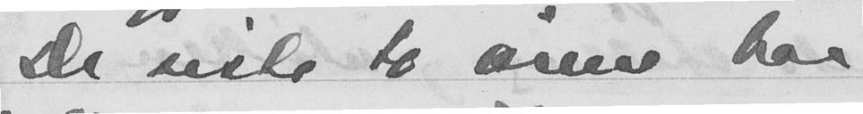De siste to årene har
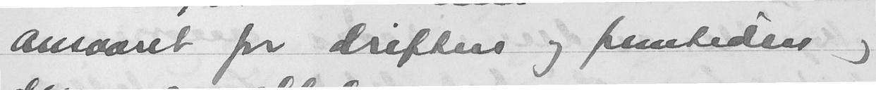ansvaret for driften og fremtiden og
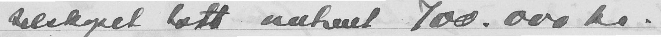selskapet hatt omtrent 700.000 kr.
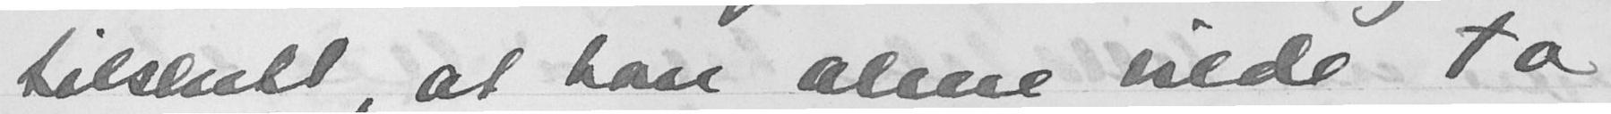tilslutt, at han alene vilde ta
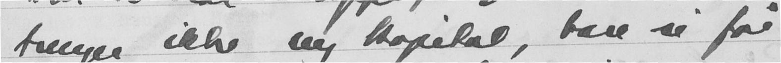trenger ikke ny kapital, bare vi får
